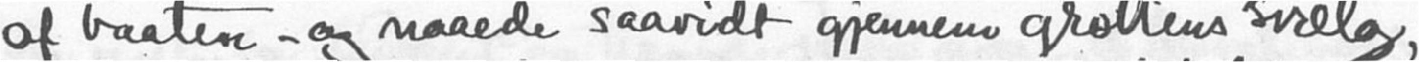af baaten - og naaede saavidt gjennem grottens svælg
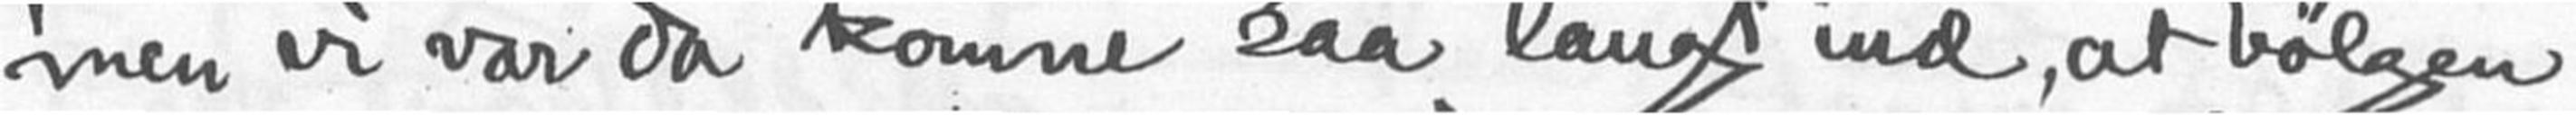men vi var da komne saa langt ind, at bølgen
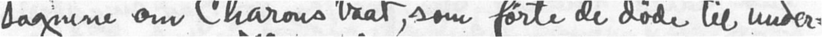sagnene om Charons baad, som førte de døde til under-
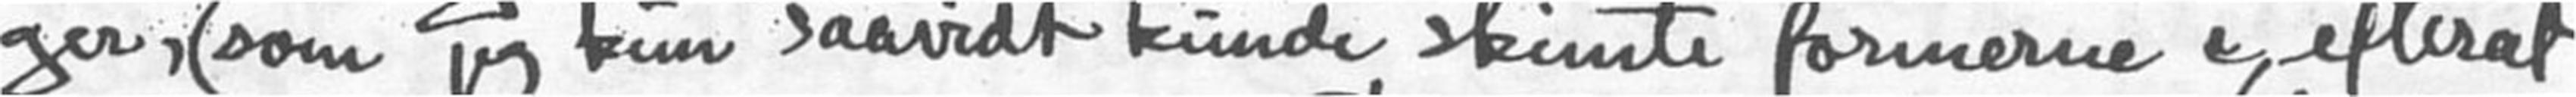ger, (som jeg kun saavidt kunde skimte formerne i, efterat
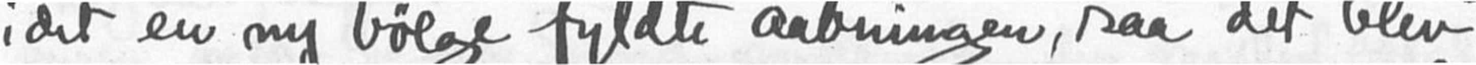idet en ny bolge fyldte aabningen, saa det blev
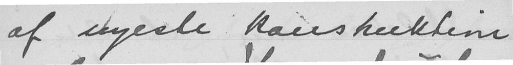af nyeste konstruktion
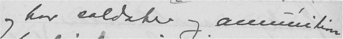og hvor soldater og amunition
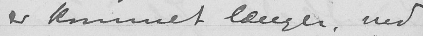er kommet længer, end
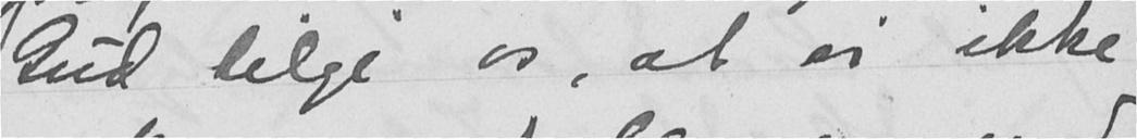Gud tilgi os, at vi ikke
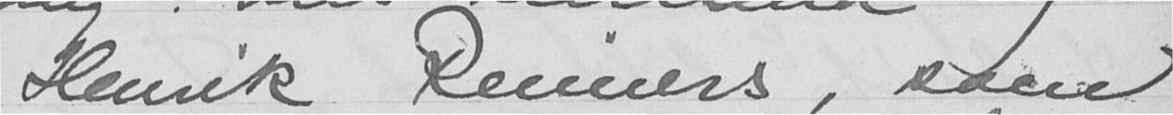Henrik Reimers, som
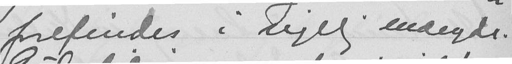forefindes i rigelig mængde.
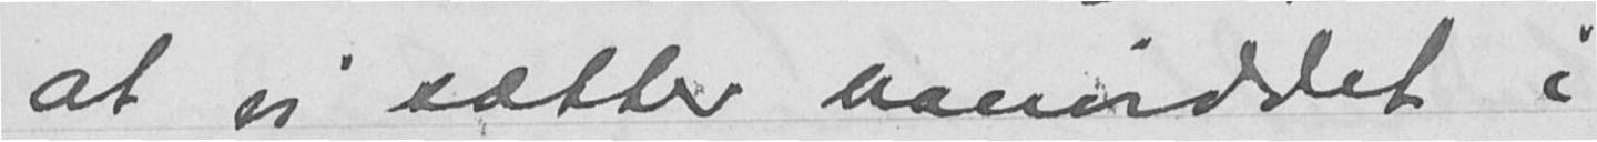at vi sætter vanviddet i
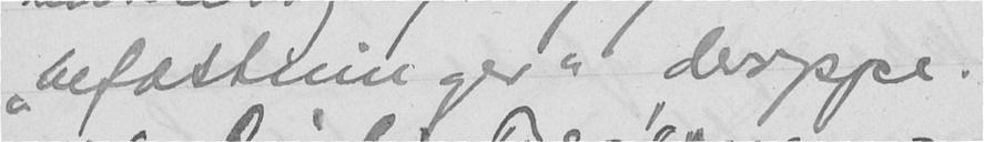"befæstninger" deroppe.
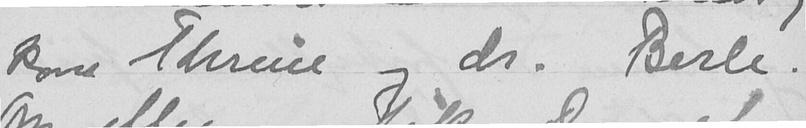kom Thrine og dr. Berle.
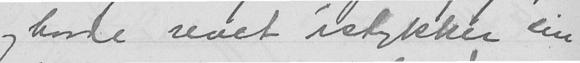og havde revet istykker sin
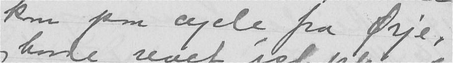kom paa cycle fra Ørje,
